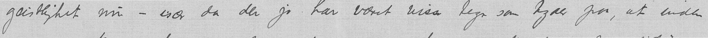geistlighet nu – især da der jo har været visse tegn som tyder paa, at inden
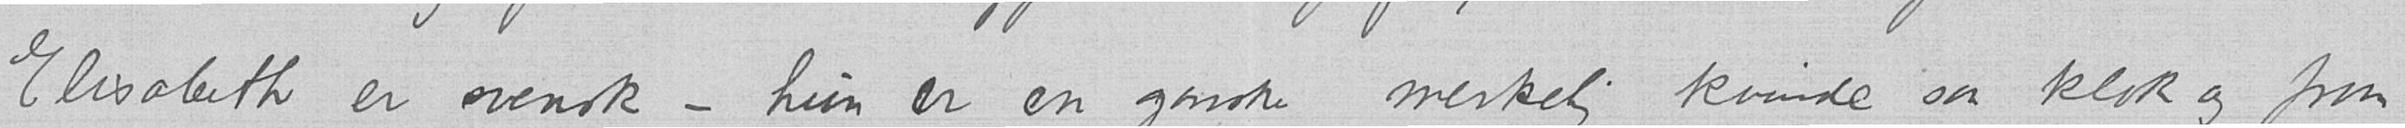Elisabeth er svensk – hun er en ganske merkelig kvinde, saa klok og from
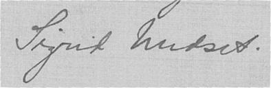Sigrid Undset.
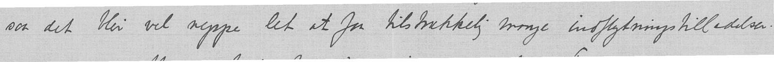saa det blir vel neppe let at faa tilstrækkelig mange indflytningstilladelser.
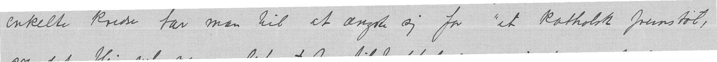enkelte kredse tar man til at ængste sig for "et katholsk fremstøt,"
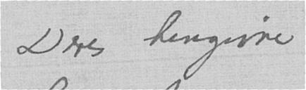Deres hengivne
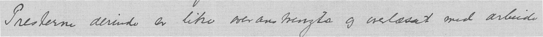Presterne derinde er like overanstrengte og overlæsset med arbeide
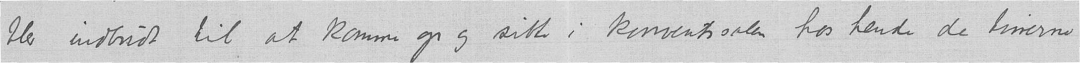blev indbudt til at komme op og sitte i konventssalen hos hende de timerne
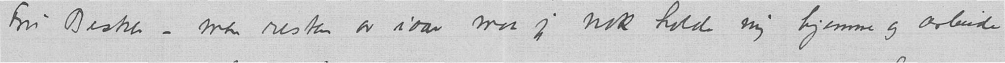Fru Backer – men resten av iaar maa jeg nok holde mig hjemme og arbeide
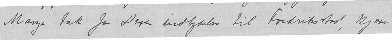Mange tak for Dere indbydelse til Fredriksstad, kjære
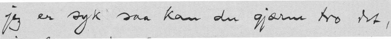jeg er syk saa kan du gjærne tro det,
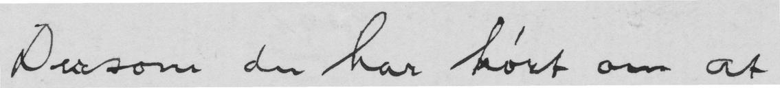Dersom du har hørt om at
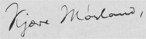Kjære Mørland,
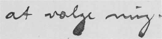at vælge mig.
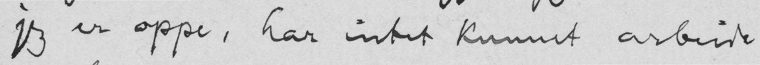jeg er oppe, har intet kunnet arbeide
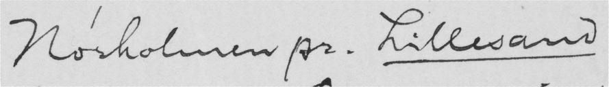Nørholmen pr. Lillesand
